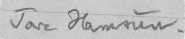Tore Hamsun.
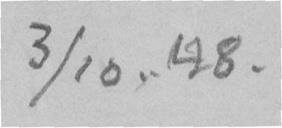3/10. 48.
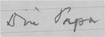Din Papa
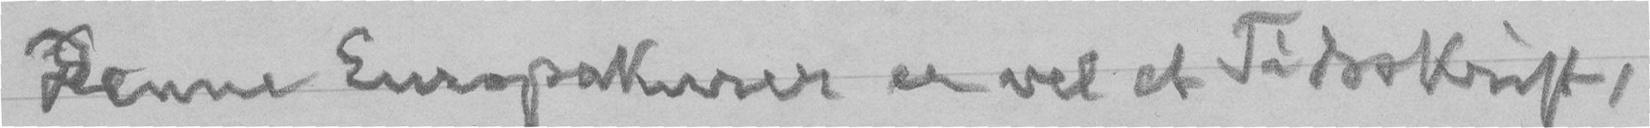Denne Europakurer er vel et Tidsskrift,
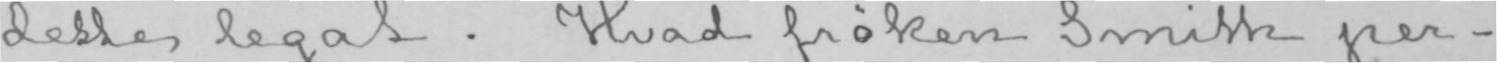dette legat. Hvad frøken Smith per-
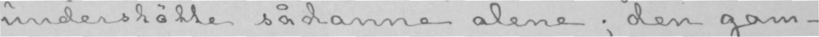understøtte sådanne alene; den gam-
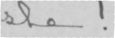ste!
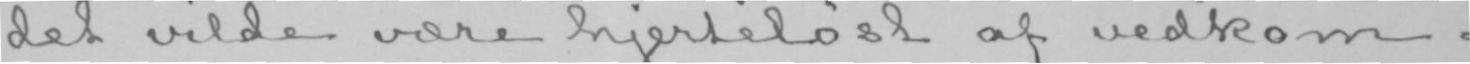det vilde være hjerteløst af vedkom-
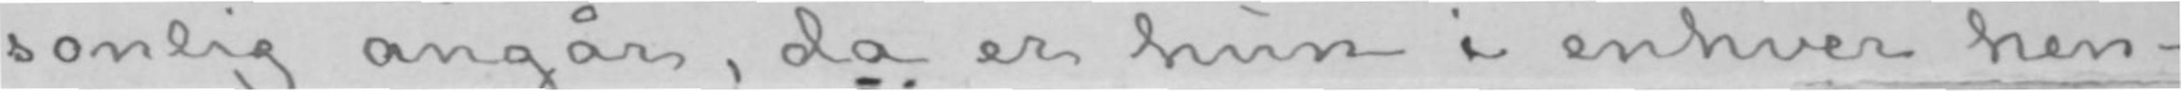sonlig angår, da er hun i enhver hen-
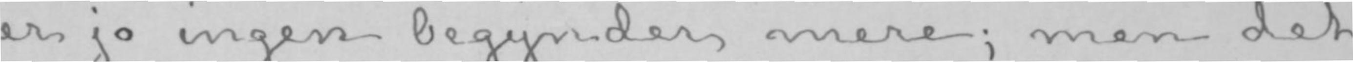er jo ingen begynder mere; men det
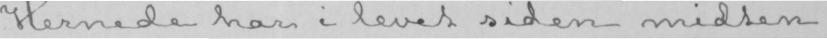Hernede har vi levet siden midten
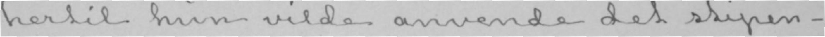hertil hun vilde anvende det stipen-
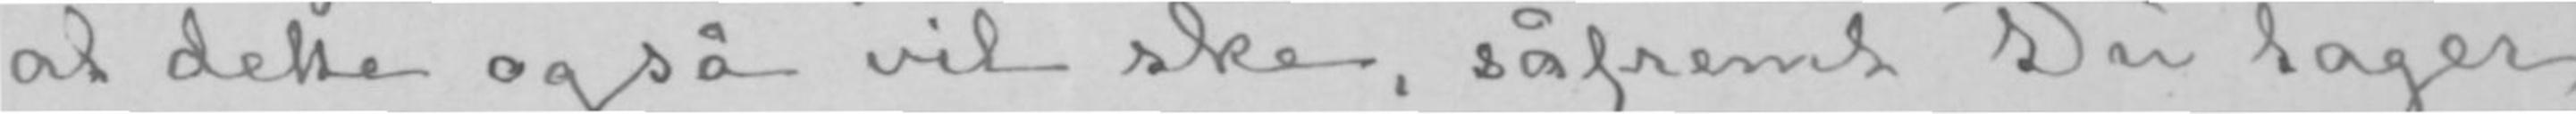at dette også vil ske, såfremt Du tager
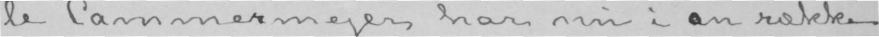le Cammermejer har nu i en række
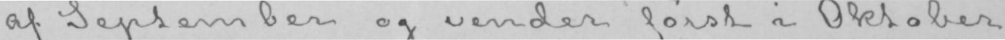af September og vender først i Oktober
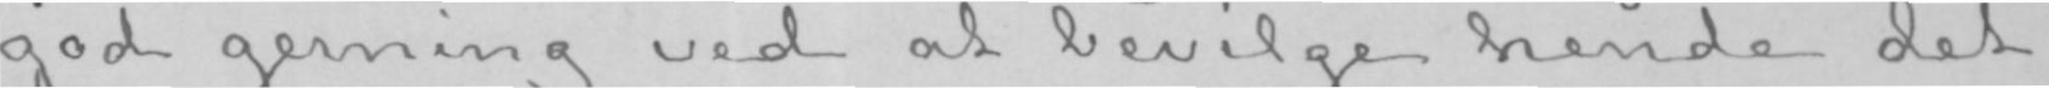god gerning ved at bevilge hende det
